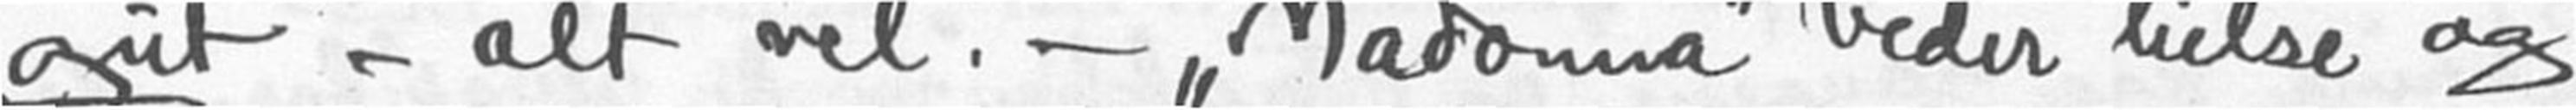gut - alt vel. - "Madonna" beder hilse og
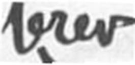brev
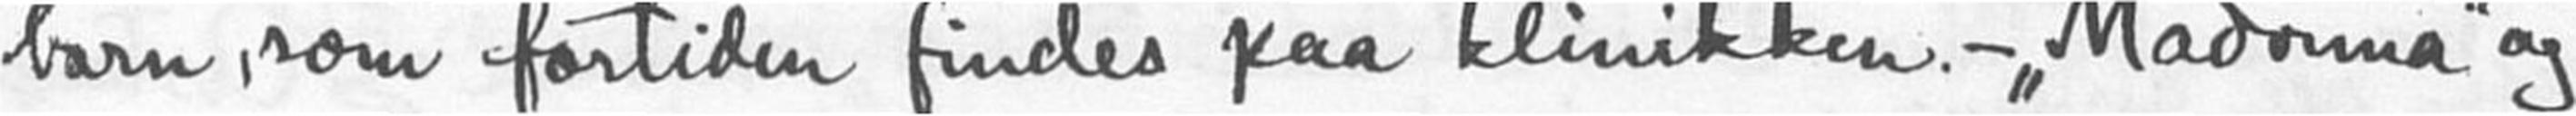barn, som fortiden findes paa klinikken. -"Madonna" og
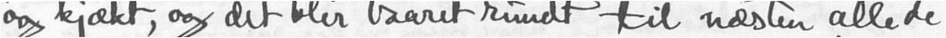og kjækt, og det blir baaret rundt til næsten alle
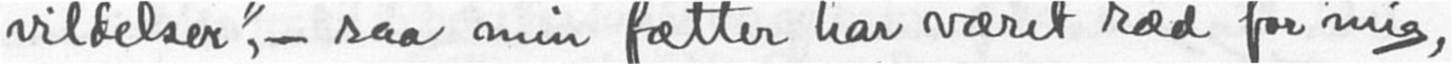vildelser", - saa min fætter har været red for mig,
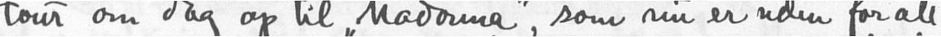tour om dag op til "Madonna", som nu er uden for
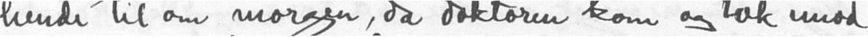hende til om morgen, da doktoren kom og tok imod
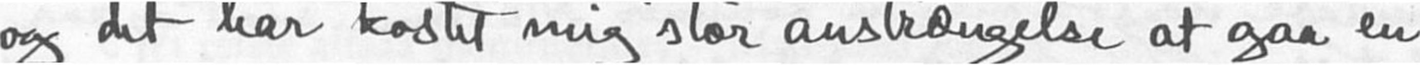og det har kostet mig stor anstrængelse at gaa en
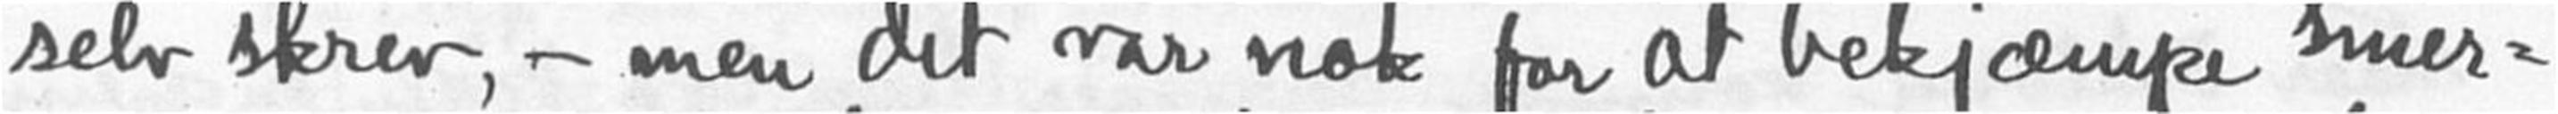selv skrev, - men det var nok for at bekjæmpe smer-
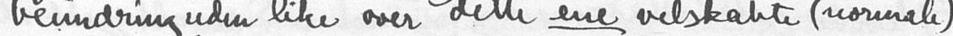beundring uden like over dette ene velskabte (normale)
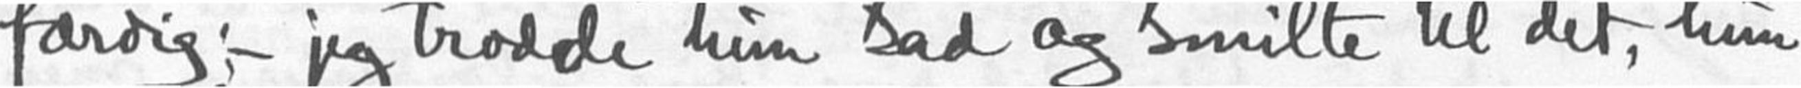færdig,- jeg trodde hun sad og smilte til det, hun
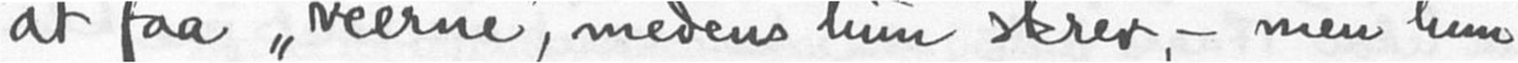at faa "veerne", medens hun skrev, - men hun
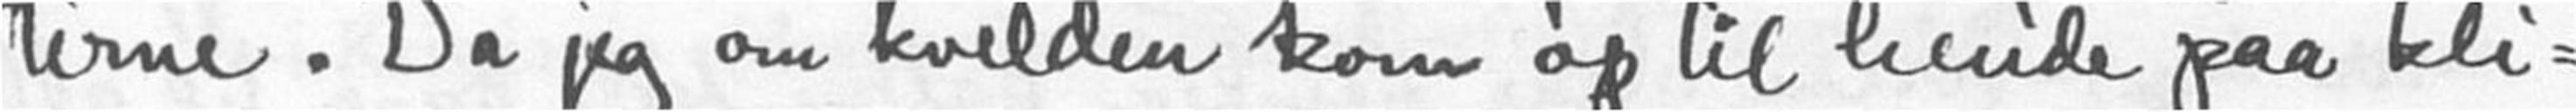terne. Da jeg om kvelden kom op til hende paa kli-
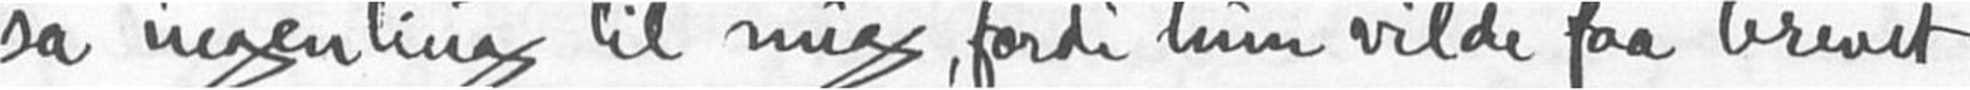sa ingenting til mig, fordi hun vilde faa brevet
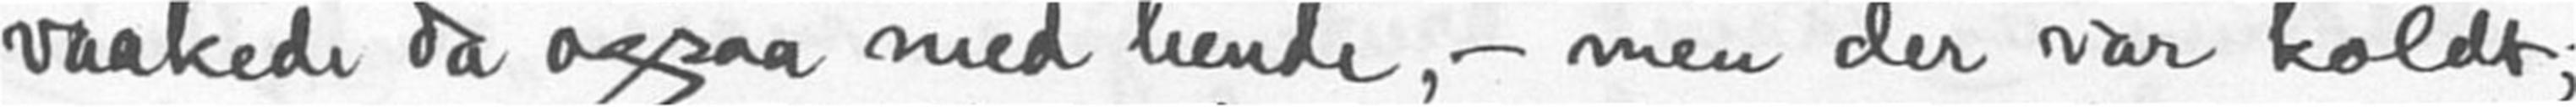vaakede da ogsaa med hende, - men der var koldt
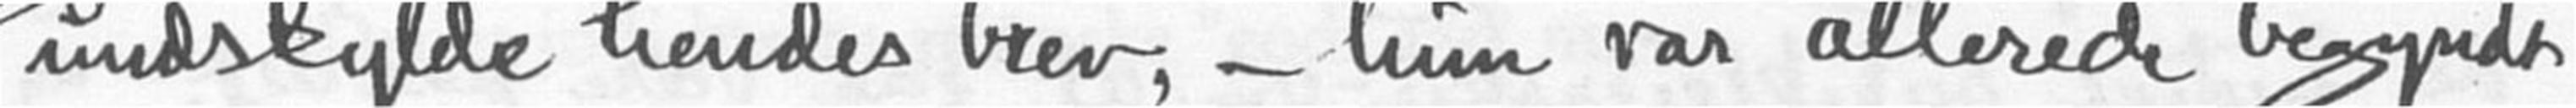undskylde hendes brev, - hun var allerede begynt
